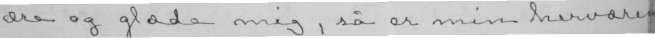ære og glæde mig, så er min herværende
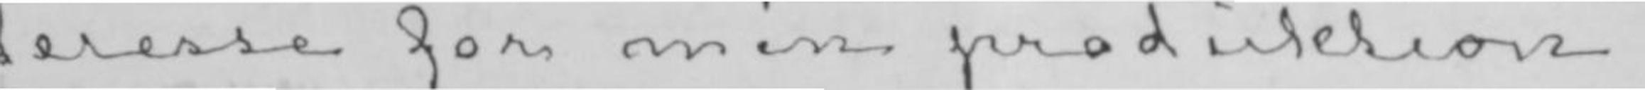teresse for min produktion.
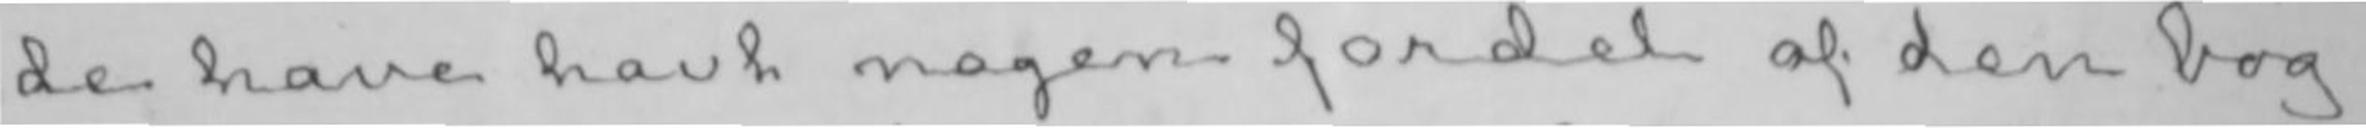de have havt nogen fordel af den bog,
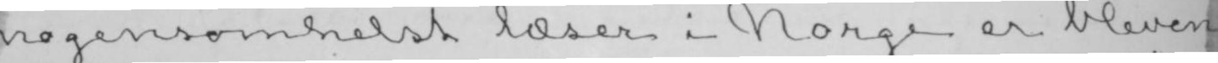nogensomhelst læser i Norge er bleven
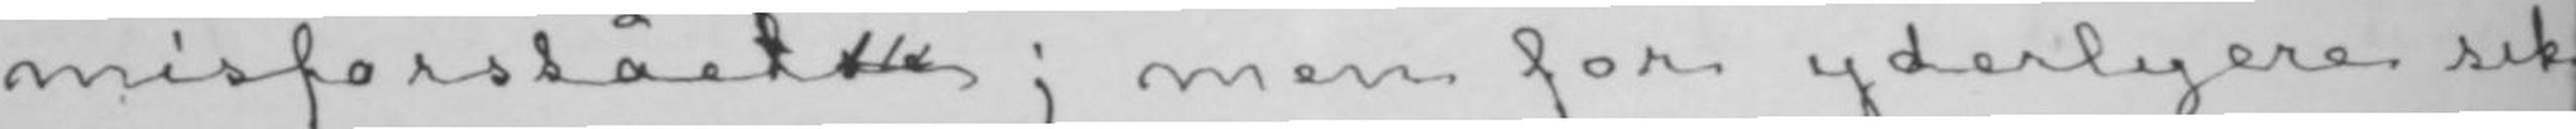misforståeltse; men for yderligere sik-
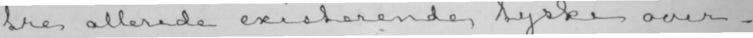tre allerede existerende tyske over-
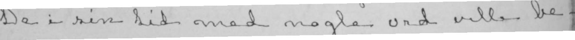De i sin tid med nogle ord ville be-
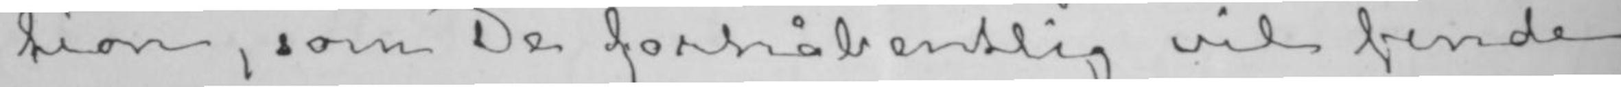tion, som De forhåbentlig vil finde
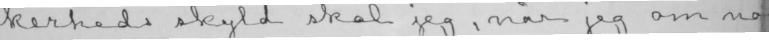kerheds skyld skal jeg, når jeg om no-
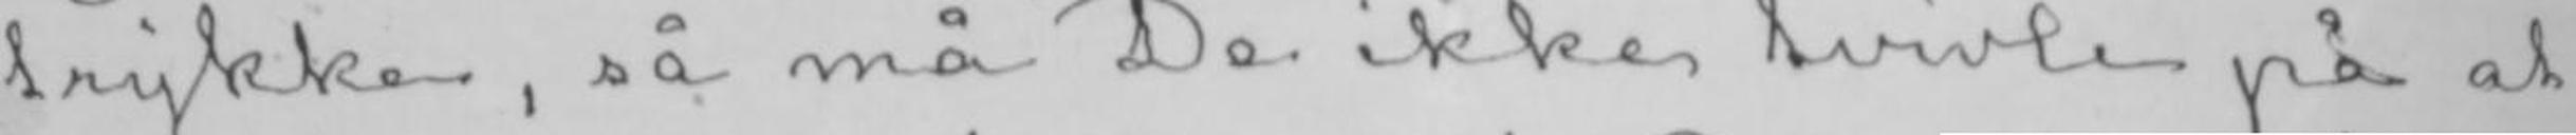trykke, så må De ikke tvivle på at
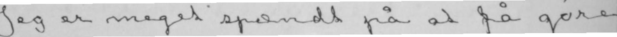Jeg er meget spændt på at få gøre
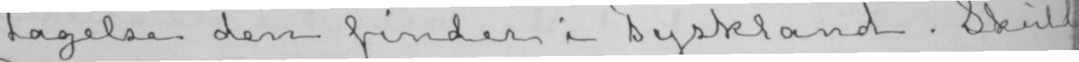tagelse den finder i Tyskland. Skulde
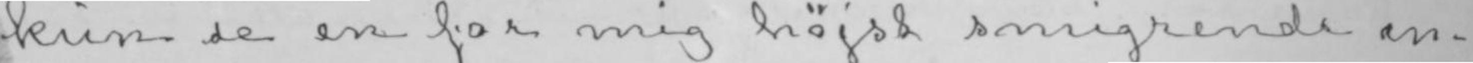kun se en for mig højst smigrende in-
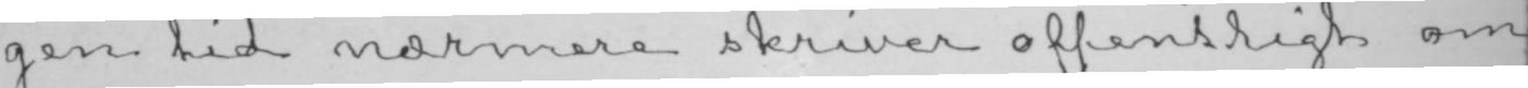gen tid nærmere skriver offentligt om
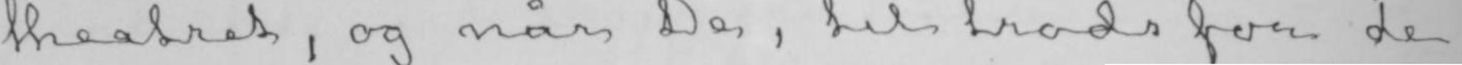theatret, og når De, til trods for de
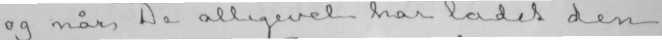og når De alligevel har ladet den
